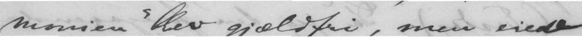monien" blev gjældfri, men eiede
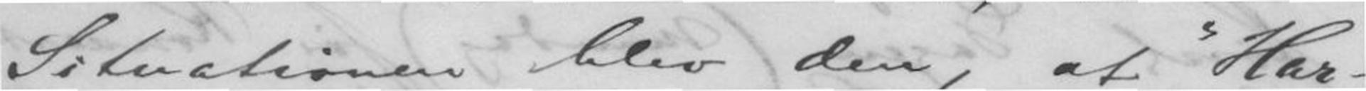Situationen blev den, at "Har-
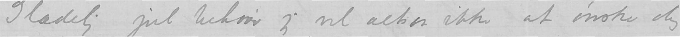Glædelig jul behøver jeg vel altsaa ikke at ønske dig,
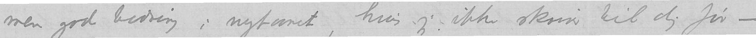men god bedring i nytaaret, hvis jeg ikke skriver til dig før –
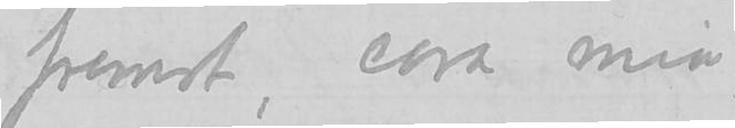fremst, cora mia,
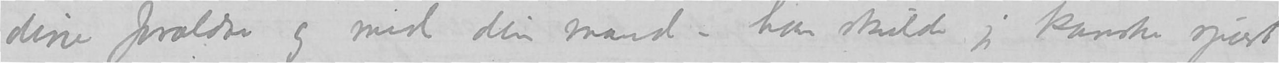dine forældre og med din mand – han skulde jeg kanske spurt
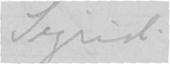Sigrid
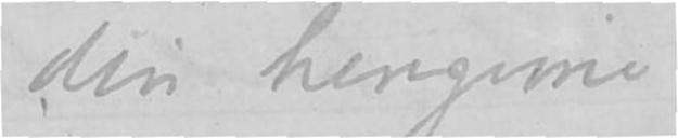din hengivne
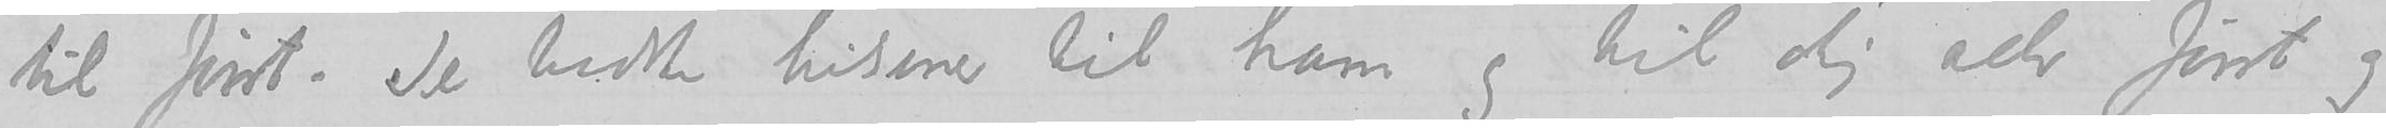til først.  De bedste hilsener til ham og til dig aller selv først og
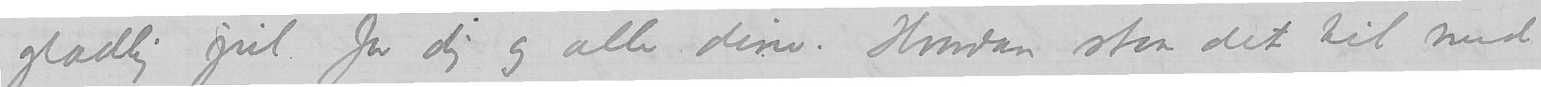glædlig jul for dig og alle dine. Hvordan staar det til med
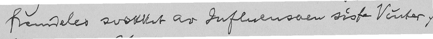fremdeles svækket av Influensaen siste Vinter,
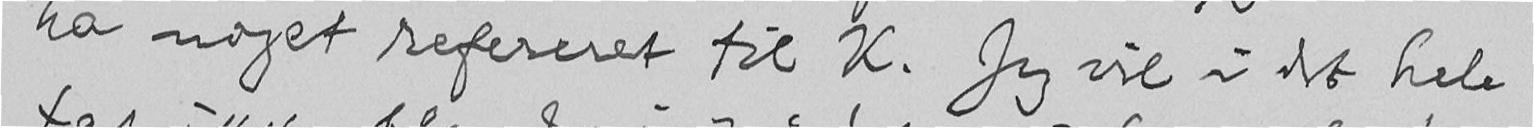ha noget refereret til K. Jeg vil i det hele
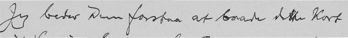Jeg beder Dem forstaa at baade dette Kort
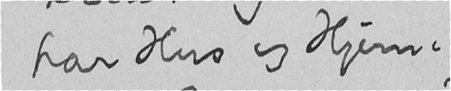har Hus og Hjem.
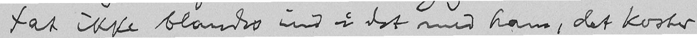tat ikke blandes ind i det med ham, det koster
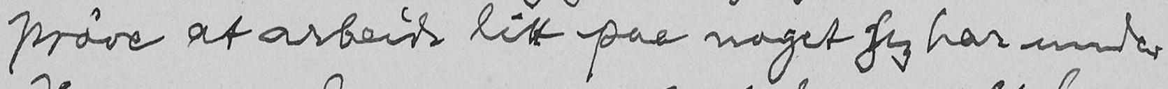prøve at arbeide litt paa noget jeg har under
